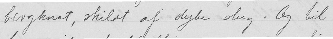bergknat, skildt af dybe slug. Og til
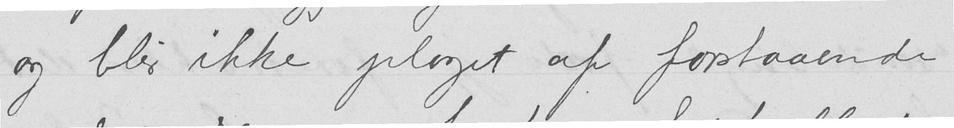og blir ikke plaget af forstaaende
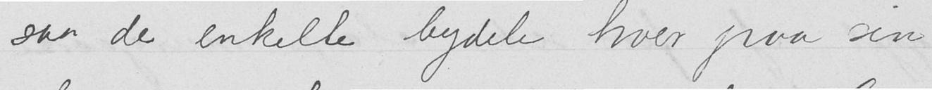saa de enkelte bydele hver paa sin
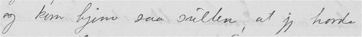og kom hjem saa sulten, at jeg havde
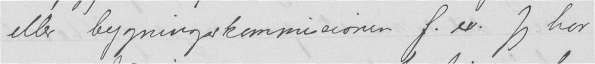eller bygningskommisionen f.ex. Jeg har
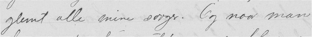glemt alle mine sorger. Og naar man
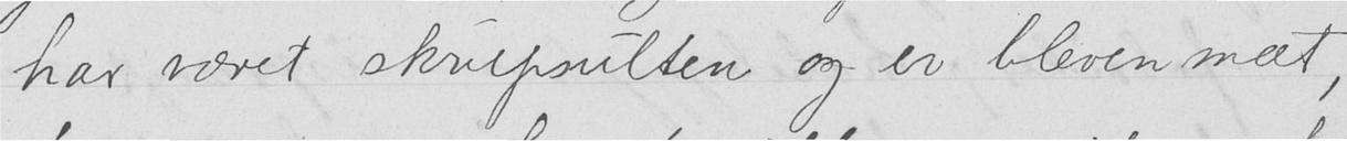har været skrupsulten og er bleven mæt,
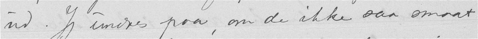ud. Jeg undres paa, om de ikke saa smaat
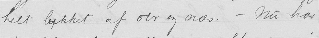helt lukket af øer og næs. - Nu har
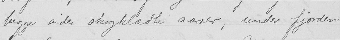begge sider skogklædte aaser, under fjorden
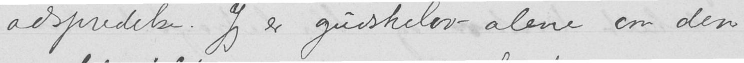adspredelse. Jeg er gudskjelov alene om den
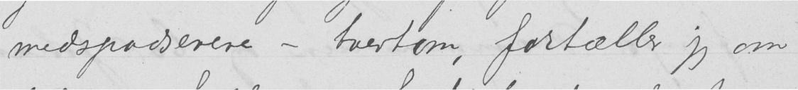medspadserere - tvertom, fortæller jeg om
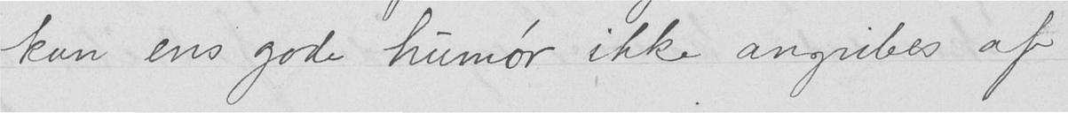kan ens gode humør ikke angribes af
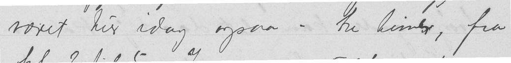været tur idag ogsaa - tre timer, fra
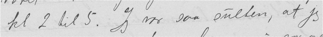kl 2 til 5. Jeg var saa sulten, at jeg
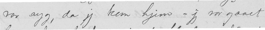var syg, da jeg kom hjem - jeg var gaaet
